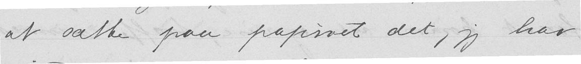at sætte paa papiret det, jeg har
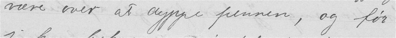være over at dyppe pennen, og før
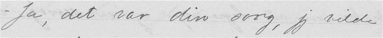- Ja, det var din sorg, jeg vilde
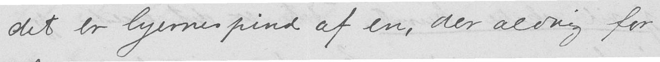det er hjernespind af en, der aldrig for
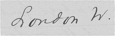London W.
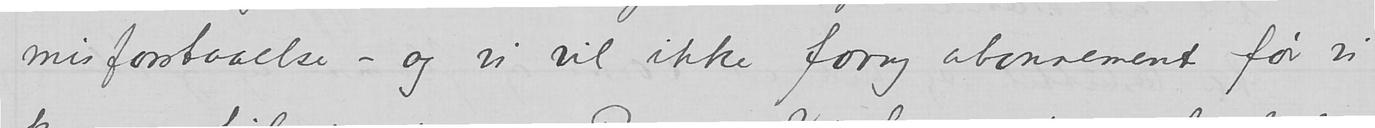misforstaaelse – og vi vil ikke forny abonnement før vi
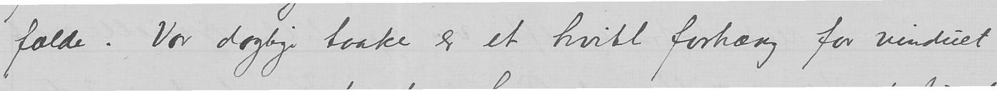fælde. Vor daglige taake er et hvitt forhæng for vinduet
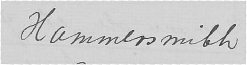Hammersmith
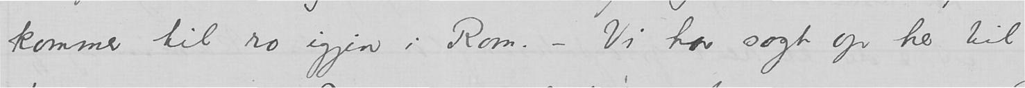kommer til ro igjen i Rom. – Vi har sagt op her til
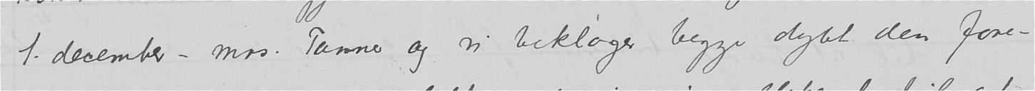1. december – mrs. Tanner og vi beklager begge dybt den fore-
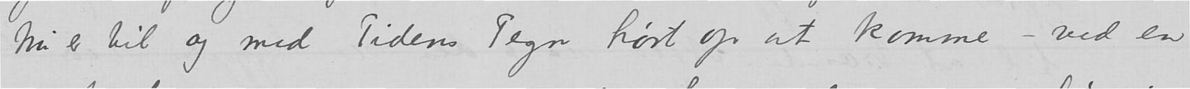Nu er til og med Tidens Tegn hørt op at komme – ved en
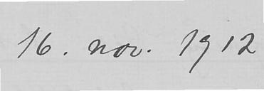16. nov. 1912
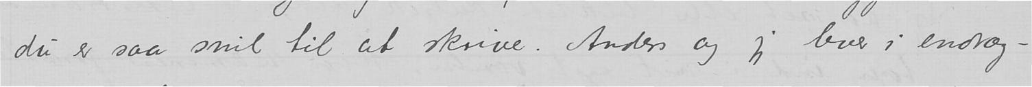skrive. Anders og jeg lever i endræg -
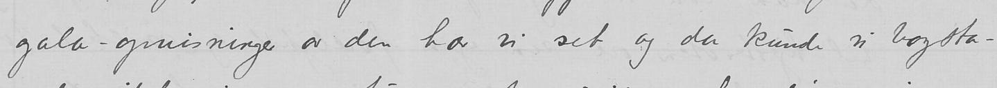gala-opvisninger av den har vi set og da kunde vi bog-
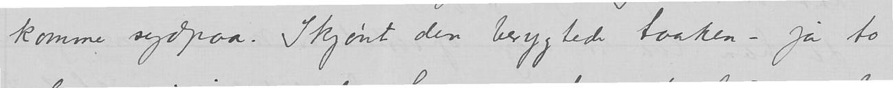komme sydpaa. Skjønt den berygtede taaken – ja to
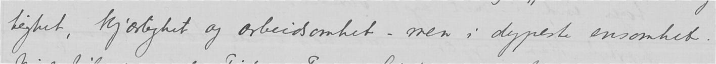tighet,  kjærlighet og arbeidsomhet – men i dypeste ensomhet.
